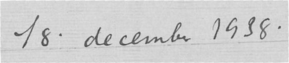18. december 1938.
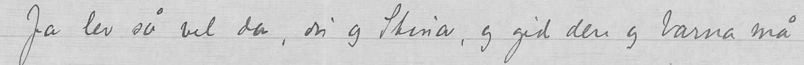Ja lev så vel da, du og Stina, og gid dere og barna må
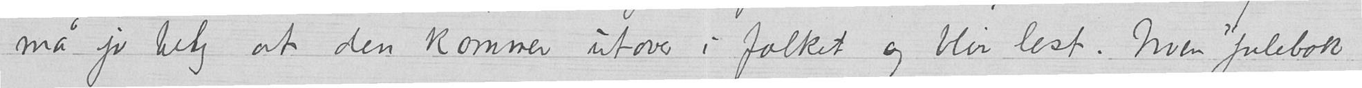må jo bety at den kommer utover i folket og blir lest. Men julebok
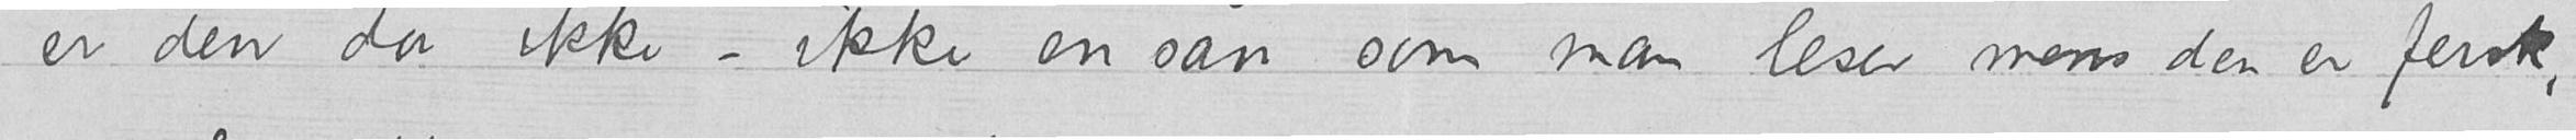er den da ikke – ikke en sån som man leser mens den er fersk,
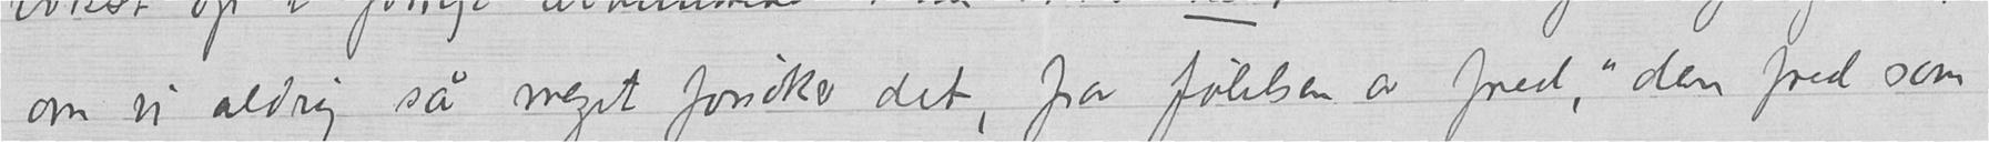om vi aldrig så meget forsøker det, fra følelsen av fred, "den fred som
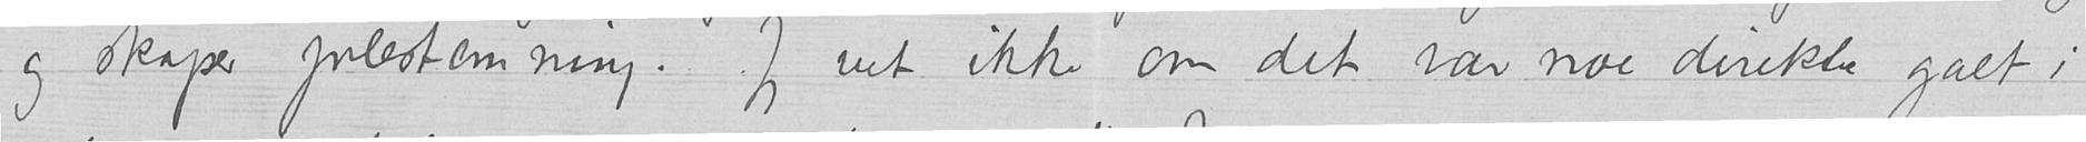og skaper julestemning. Jeg vet ikke om det var noe direkte galt i
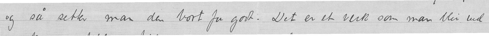og så setter man den bort for godt. Det er et verk som man blir ved
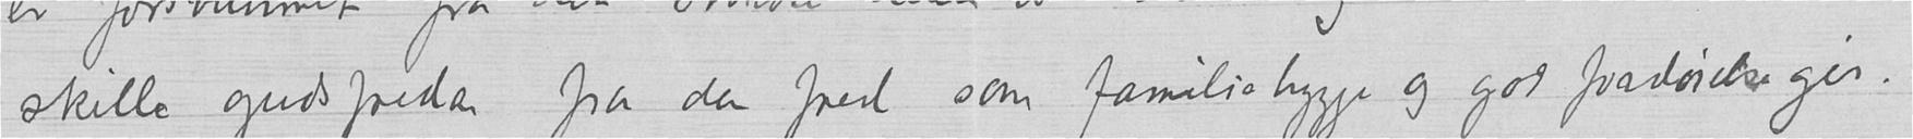skille gudsfreden fra den fred som familiehygge og god fordøielse gir.
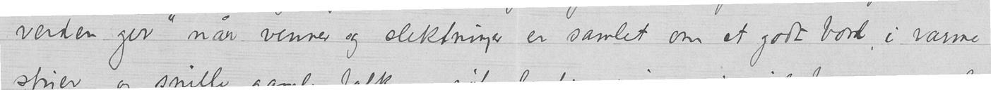verden gir" når venner og slektninger er samlet om et godt bord i varme
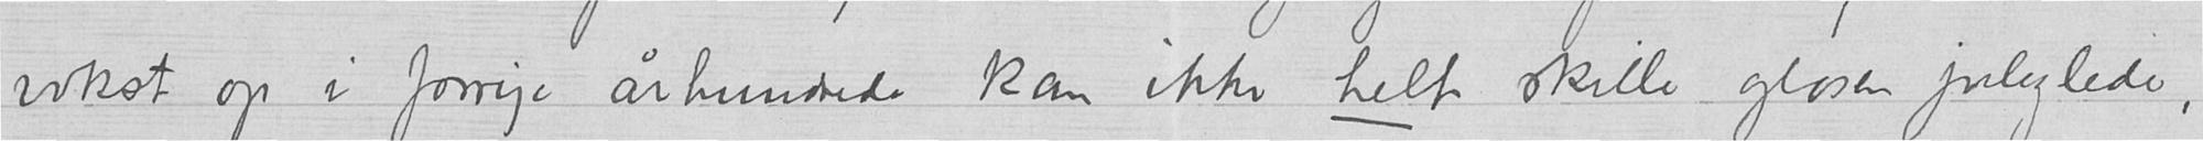vokst op i forrige århundrede kan ikke helt skille glosen juleglede,
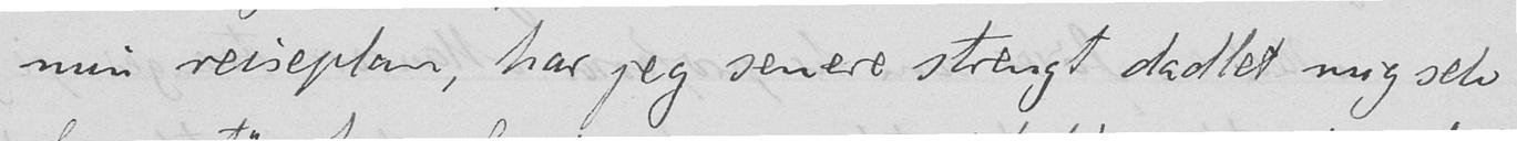min reiseplan, har jeg senere strengt dadlet mig selv
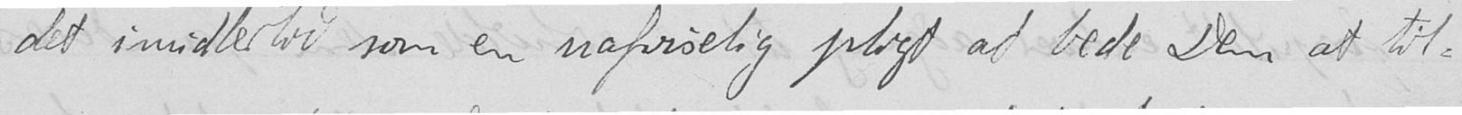det imidlertid som en uafviselig pligt at bede Dem at til-
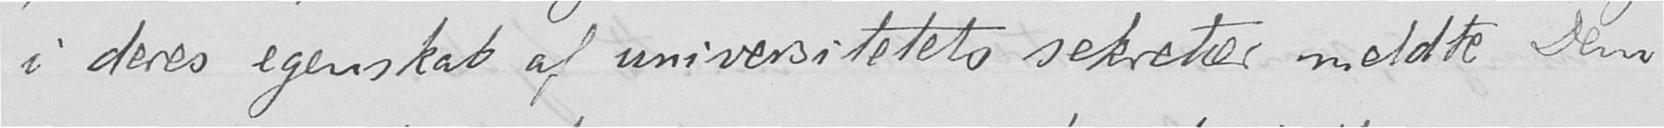i deres egenskab af universitetets sekretær meldte Dem
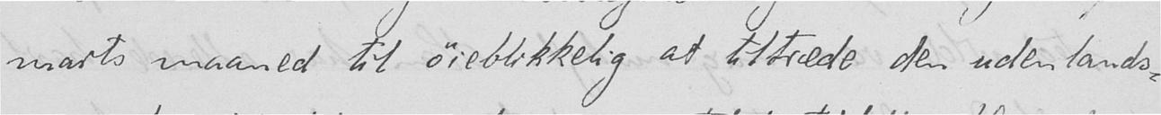marts maaned til øieblikkelig at tiltræde den udenlands-
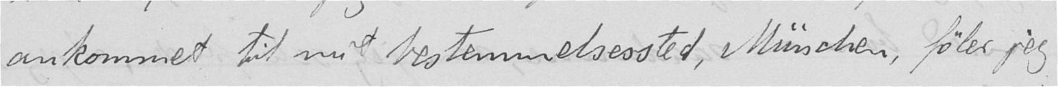ankommet til mit bestemmelsessted, München, føler jeg
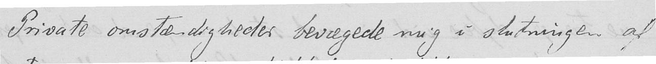Private omstændigheder bevægede mig i slutningen af
